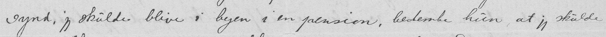synd, jeg skulde blive i byen i en pension, bestemte hun, at jeg skulde
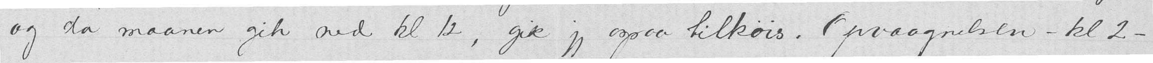og da maanen gik ned kl 12, gik jeg ogsaa tilkøis. Opvaagnelsen - kl 2 -
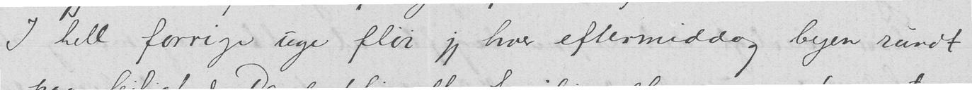I hele forrige uge fløi jeg hver eftermiddag byen rundt
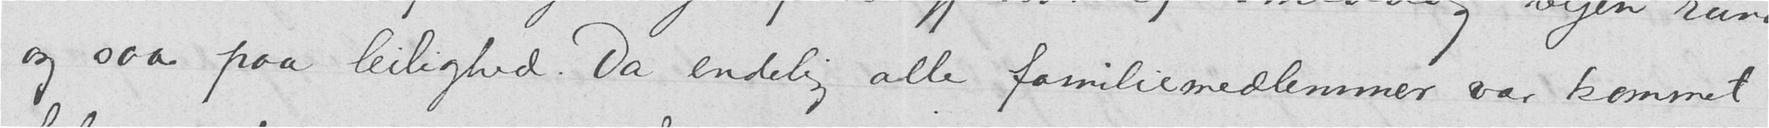og saa paa leilighed. Da endelig alle familiemedlemmer var kommet
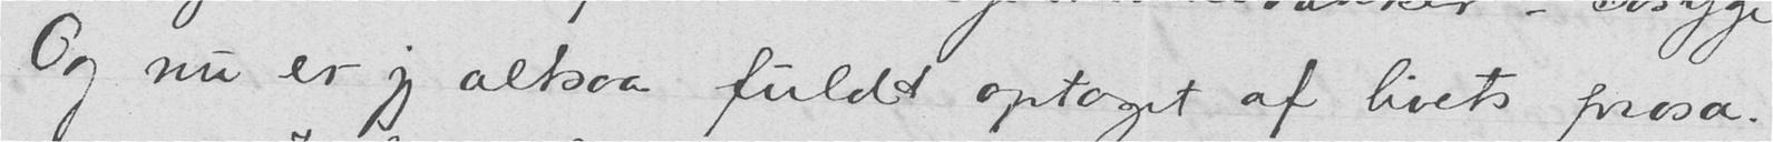Og nu er jeg altsaa fuldt optaget af livets prosa.
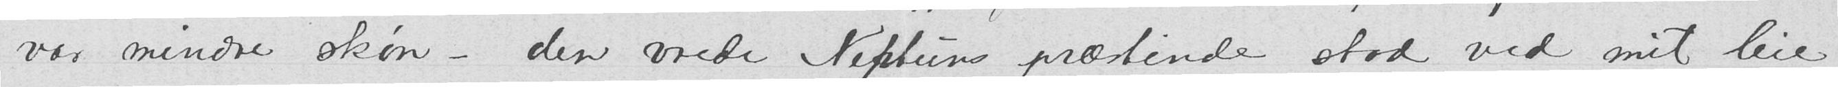var mindre skøn - den vrede Neptuns præstinde stod ved mit leie
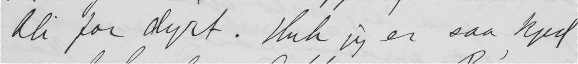bli for dyrt. Huh jeg er saa kjed
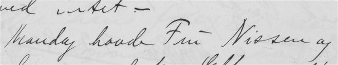Mandag havde Fru Nissen og
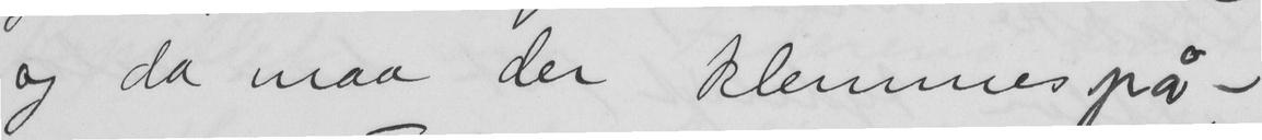og da maa der klemmes på -
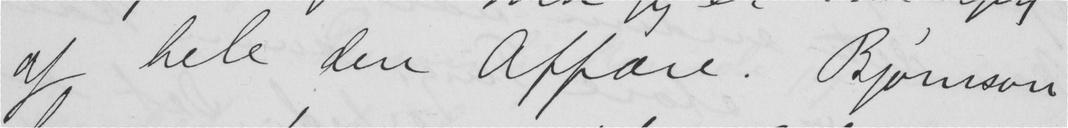af hele den Affære. Bjørnson
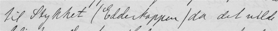til Stykket (Edderkoppen) da det vilde
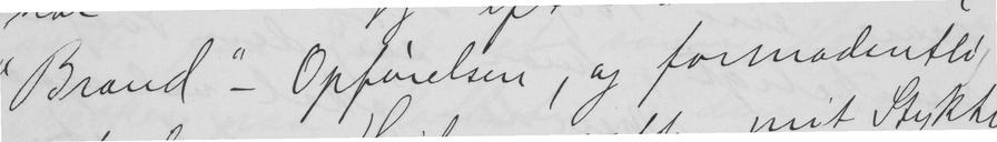"Brand" - Opførelsen, og formodentlig
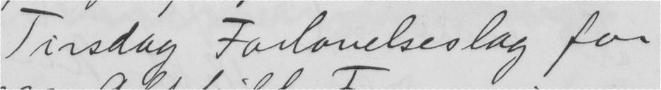Tirsdag Forlovelseslag for
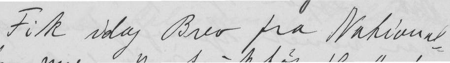Fik idag Brev fra National-
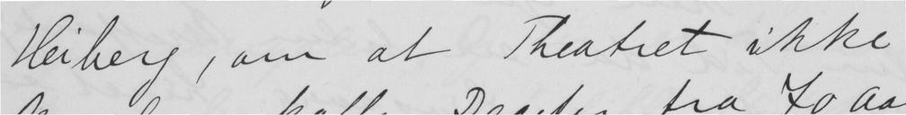Heiberg, om at Theatret ikke
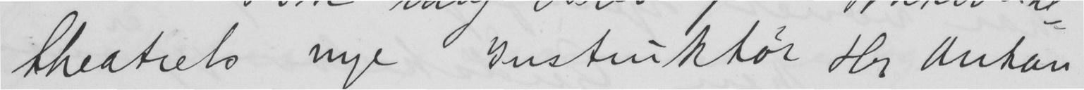theatrets nye Instruktør Hr Anton
